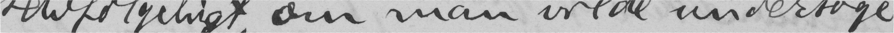selfølgeligt, om man vilde undersøge
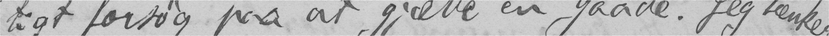tigt forsøg paa at gjætte en gaade. Jeg tænker
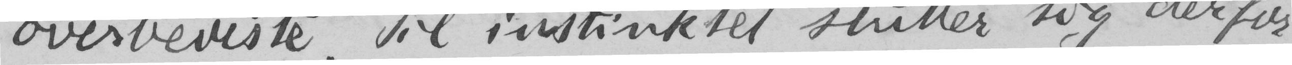overbeviste. Til instinktet slutter sig derfor
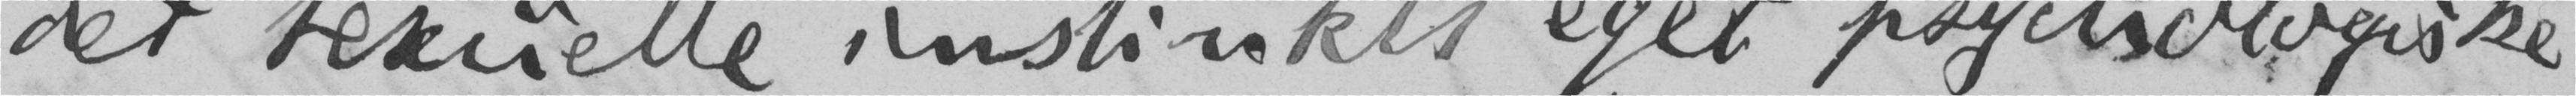det sexuelle instinkts eget psychologiske
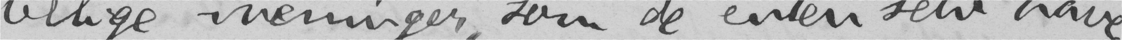belige meninger, som de enten selv have
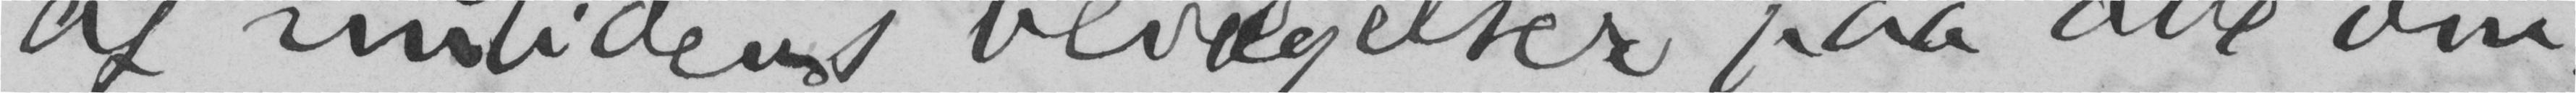af nutidens bevægelser paa alle om-
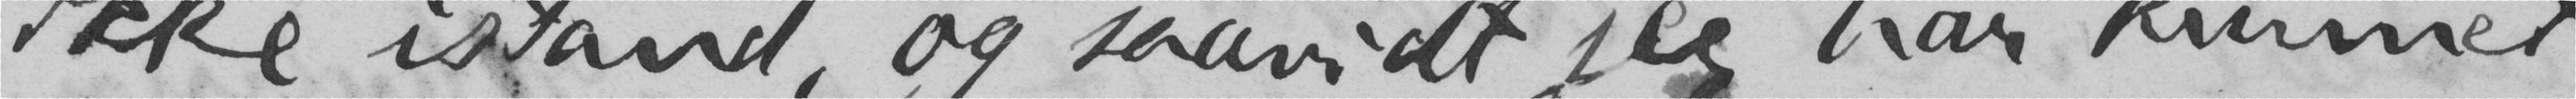ikke istand, og saavidt jeg har kunnet
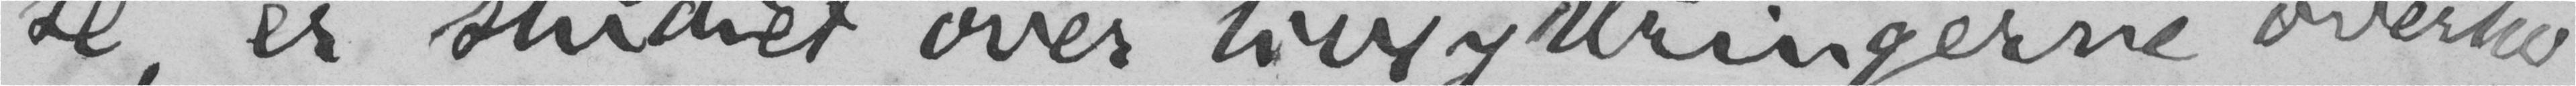se, er studiet over livsyttringerne overho-
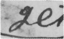get
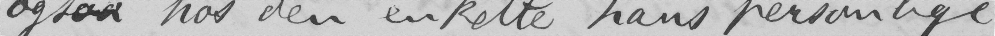ogsaa hos den enkelte hans personlige
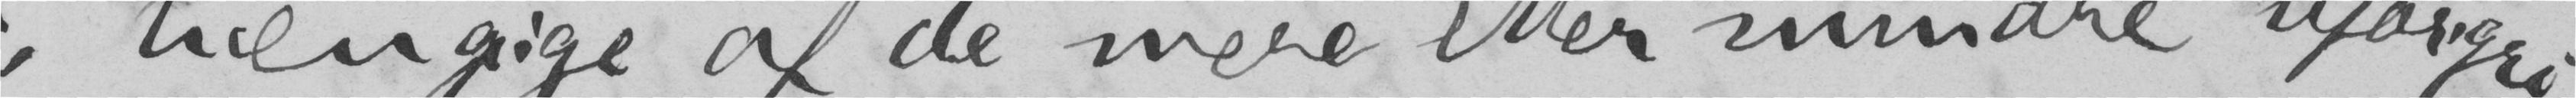hængige af de mere eller mindre uforgri-
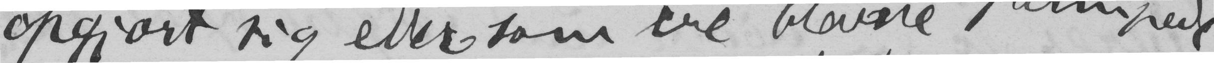opgjort sig eller som ere blevne pumpede
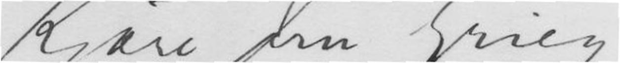Kjære fru Grieg
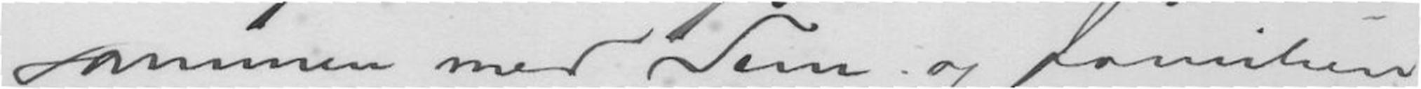sammen med Dem og familien
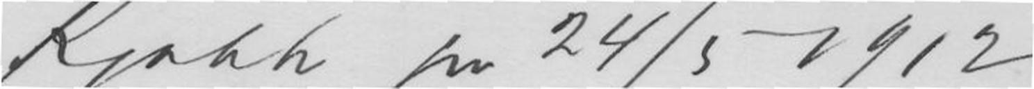Kjøbh pr 24/5 12
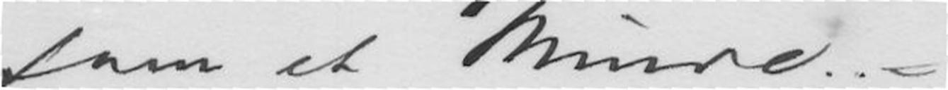som et Minde. -
